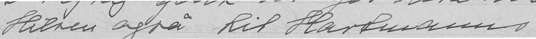Hulsen ogsa til Hartmanns
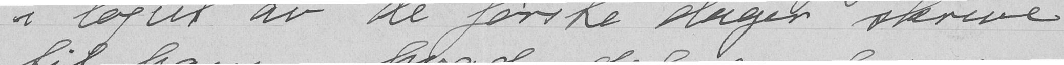i løpet av de første dager skrive
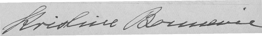Kristine Bonnvie
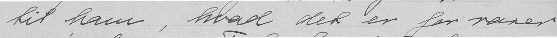til ham; hvad det er for raser
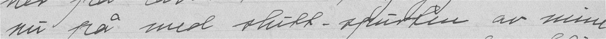nu på med slutt-spurten av mine
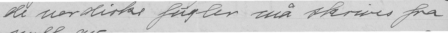de nordiske fugler må skrives fra
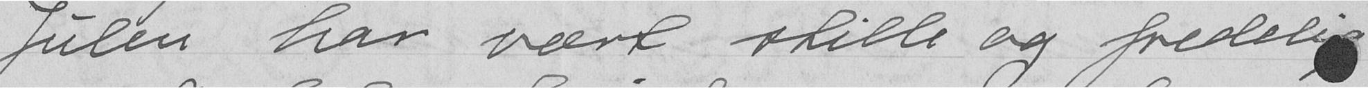Julen har vært stille og fredelig
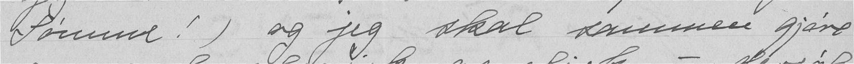Sømme!) og jeg skal sammen gjøre
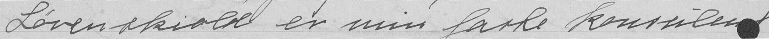Løvenskiold er min faste Konsulent
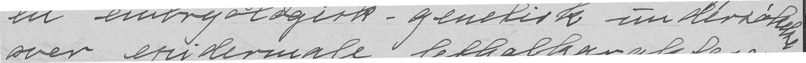en embryologisk-genetisk undersøkelse
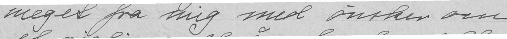meget fra mig med ønsker om
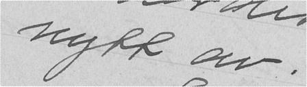nytt av.
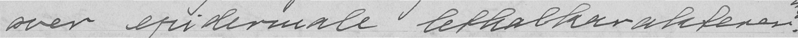over epidermale lethalkarakterer.
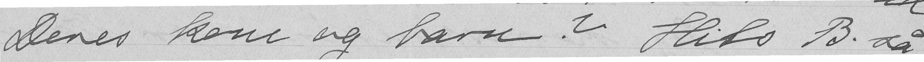Deres kone og barn? Hils B. så
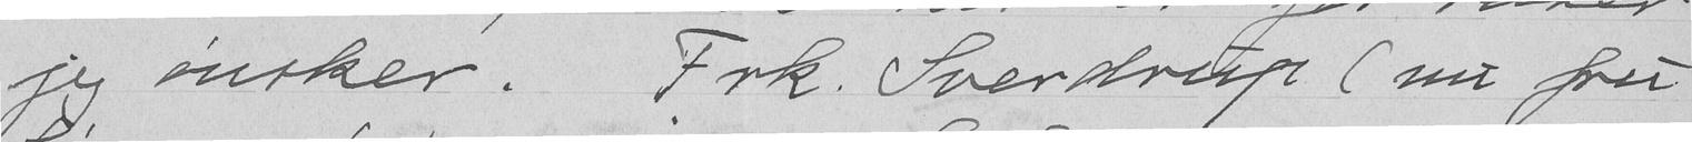jeg ønsker. Frk. Sverdrup (nu fru
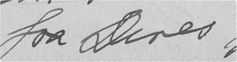fra Deres
